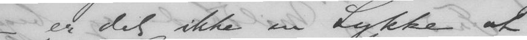- er det ikke en Lykke at
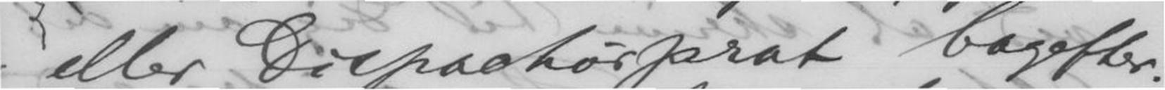eller Dispachørprat bagefter.
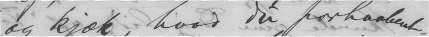og kjæk, hvad du forhaabent-
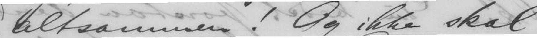Altsammen! og ikke skal
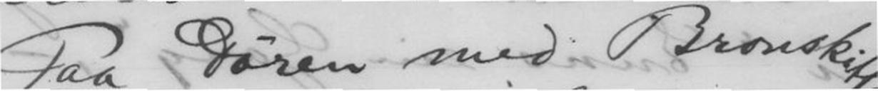Paa Døren med Bronskitten!
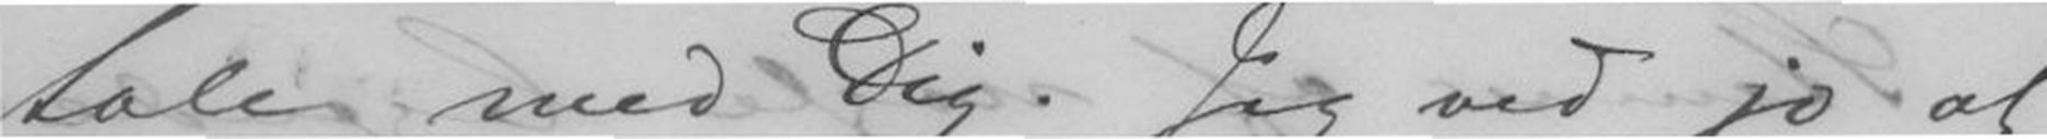tale med Dig. Jeg ved jo at
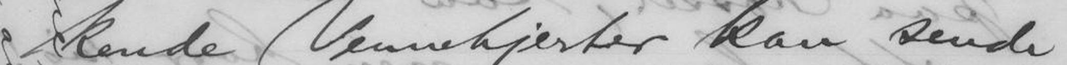kende Vennehjerter kan sende
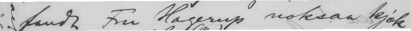fandt Fru Hagerup noksaa kjæk
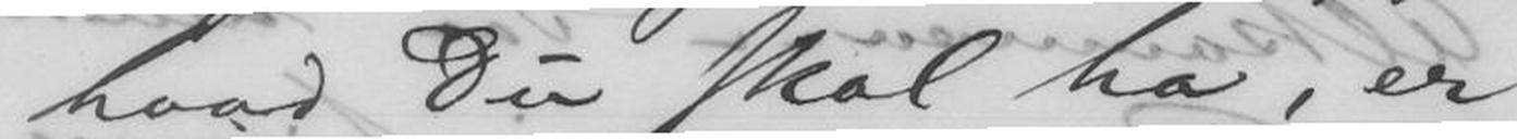hvad Du skal ha, er
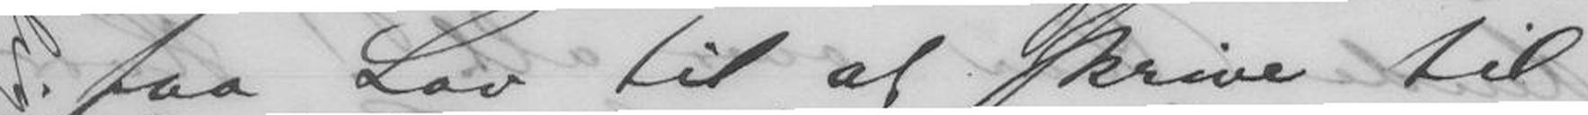faa Lov til at skrive til
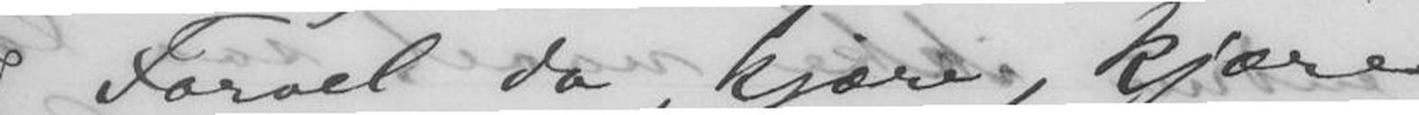Farvel da, kjære, kjære
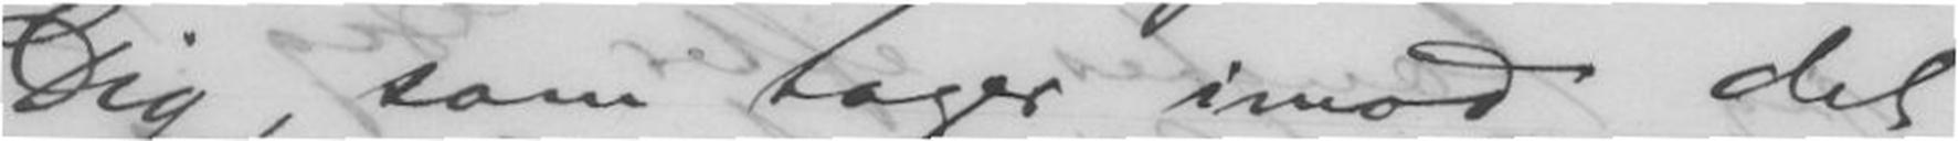Dig, som tager imod det
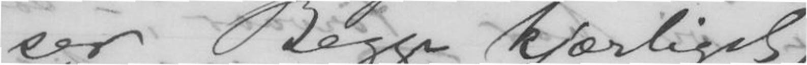ser Begge kjærligst, -
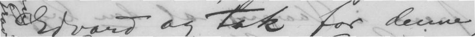Edvard og Tak for denne
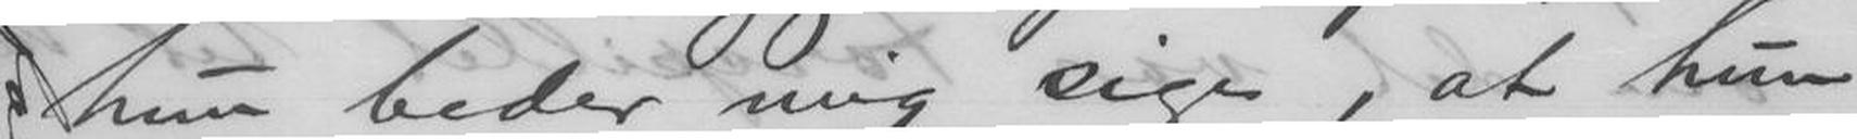hun beder mig sige, at hun
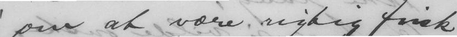om at være rigtig frisk
